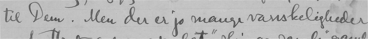til Dem. Men der er jo mange vanskeligheder
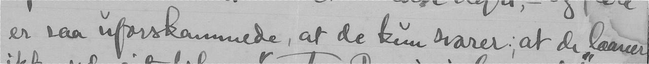er saa uforskammede, at de kun svarer; at de "laaner
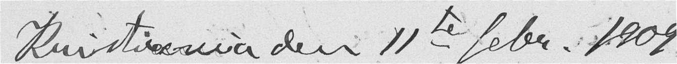Kristiania den 11te febr. 1909.
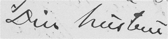Din hustru
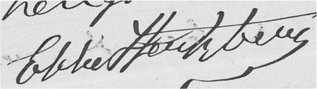Ebbe Hertzberg.
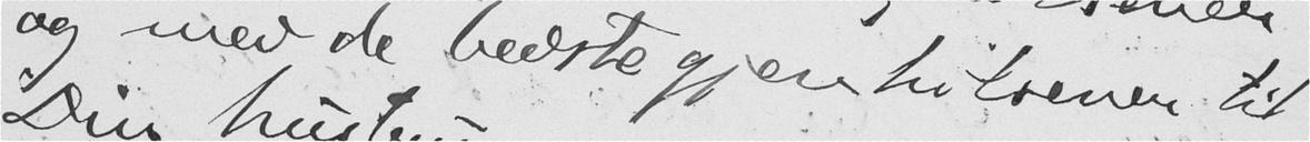og med de bedste gjenhilsener til
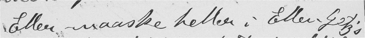Eller maaske heller i Ellen Getz's
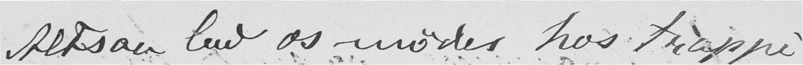Altsaa lad os mødes hos trappi-
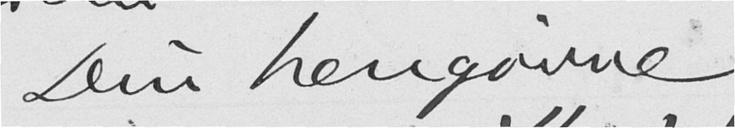Din hengivne
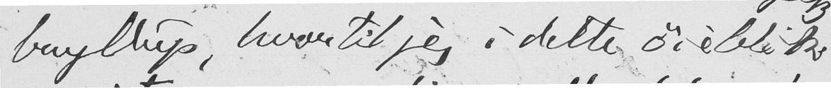bryllup, hvortil jeg i dette øieblik
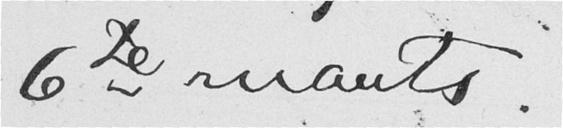6te marts.
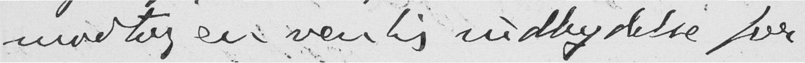modtog en venlig indbydelse for
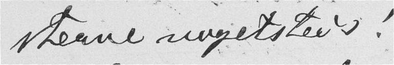sterne nogetsteds!
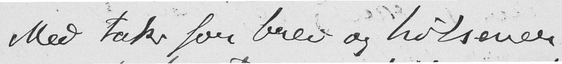Med tak for brev og hilsener
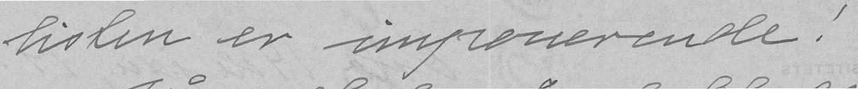listen er imponerende!
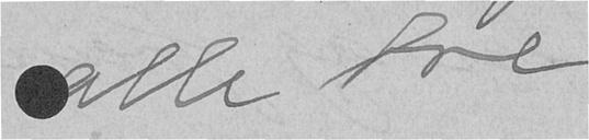alle tre
